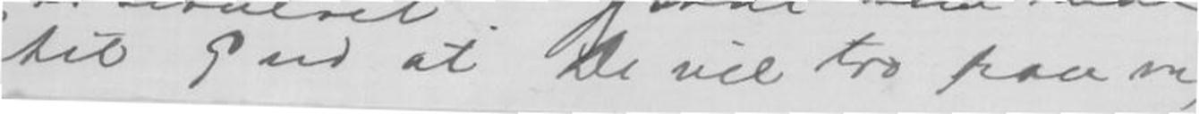til Gud at De vil tro paa mig
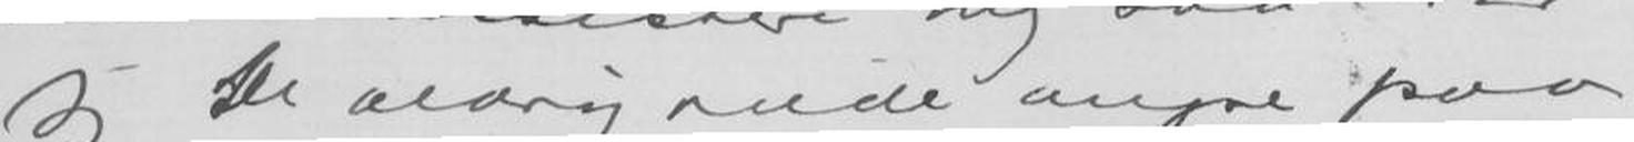jeg De aldrig vilde angre paa
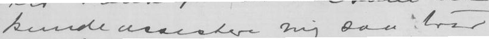kunde assistere mig saa tror
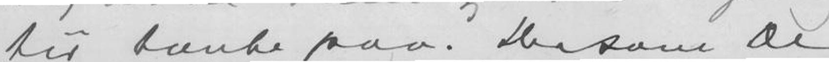tør tænke paa. Dersom De
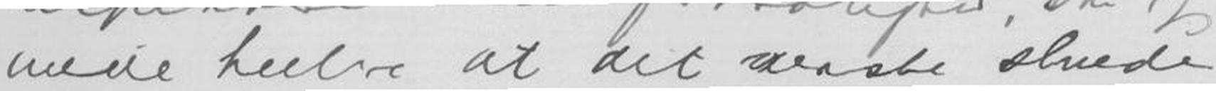vilde hellre at det wæste skulde
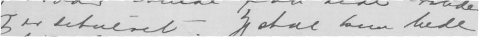jeg er situeret. Jeg skal kun bede
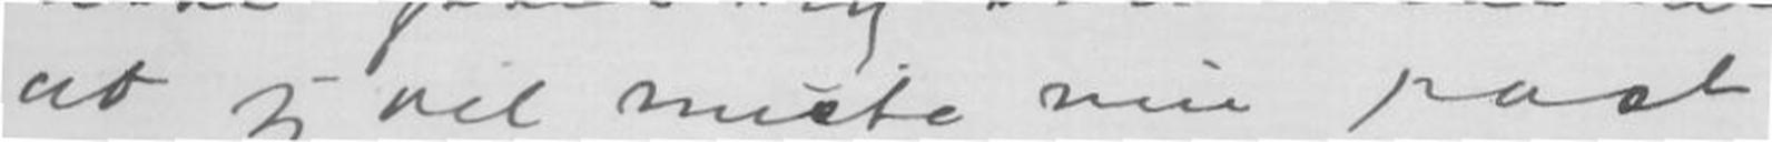at jeg vil miste min post,
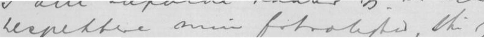respektere min fortrolighed thi jeg
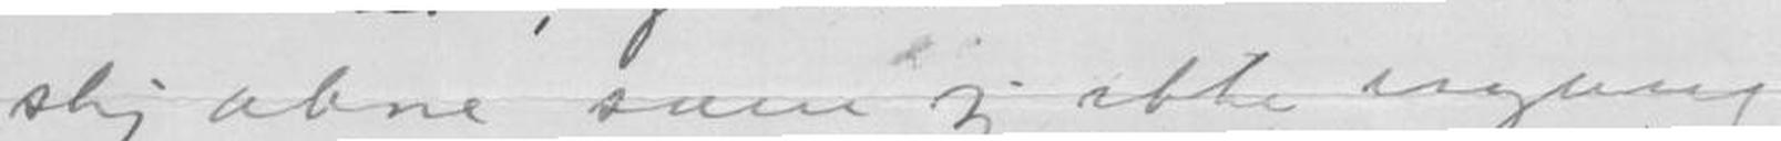skjæbne som jeg ikke engang
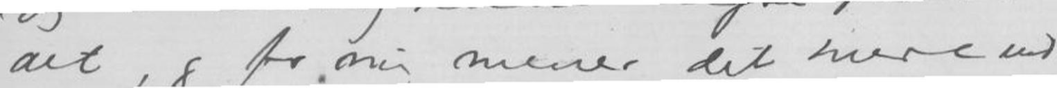det, og for mig mener det mere end
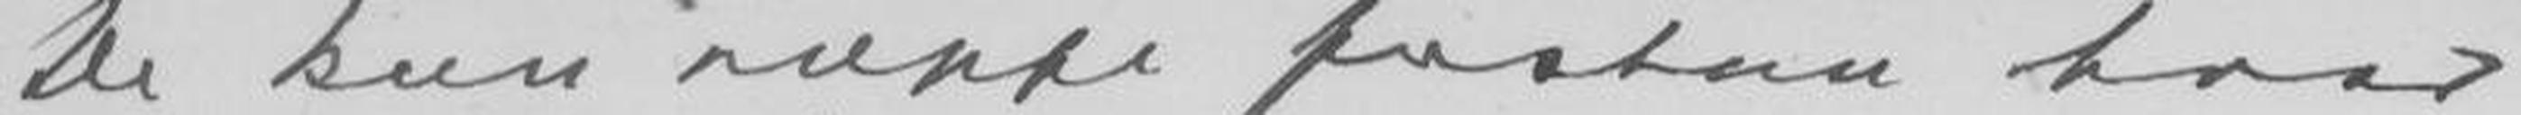De kan neppe forstaa hvor
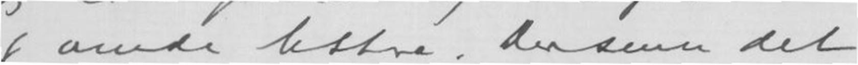og aande lettere. Dersom det
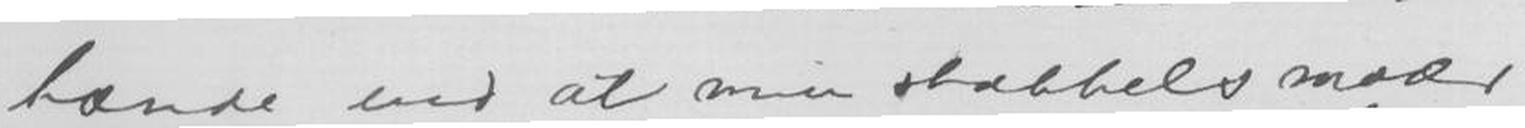hænde end at min stakkels moder
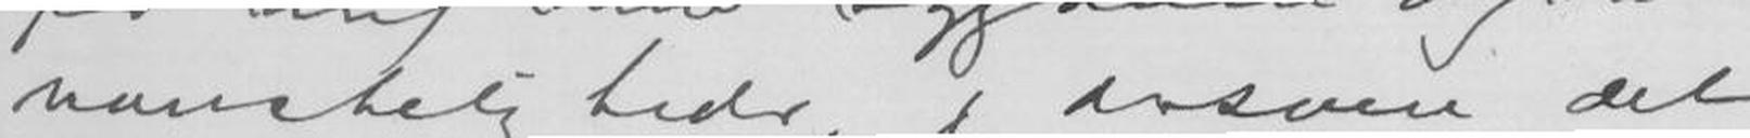vanskelig tider, og dersom det
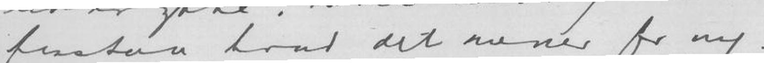forstaa hvad det mener for mig.
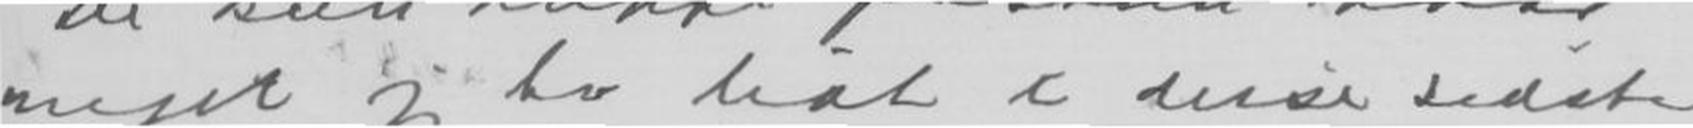meget jeg har lidt i disse sidste
